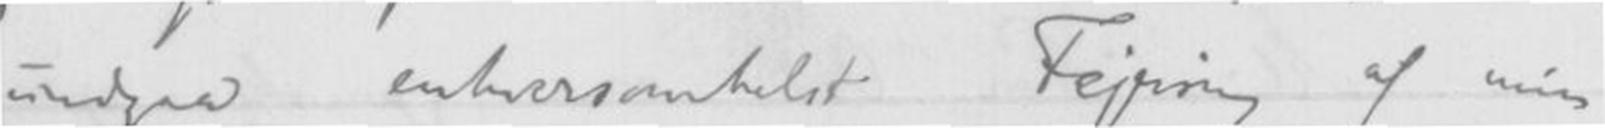undgaa enhversomhelst Fejring af min
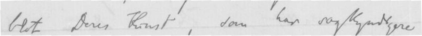blot Deres Kunst, som har sagkyndigere
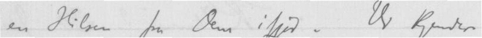en Hilsen fra Dem ifjor. Vi kjender
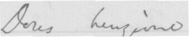Deres hengivne
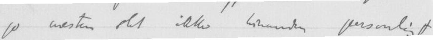jo nesten slet ikke hinanden personligt,
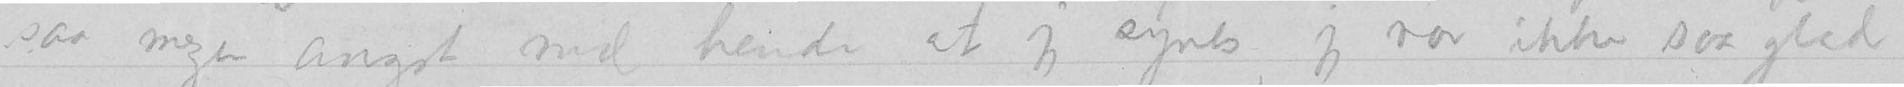saa megen/mye angst med hende at jeg synes, jeg var ikke saa glad
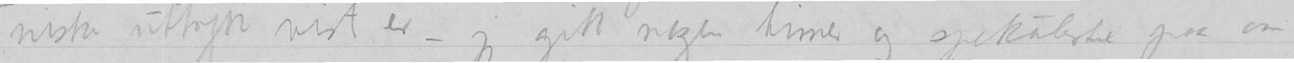niske uttryk vist er – jeg gik nogen timer og spekulerte paa om
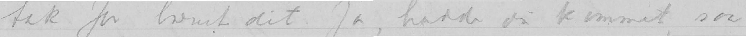tak for brevet dit. Ja, hadde du kommet, saa
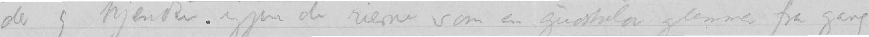der og kjendte igjen de rierne som en gudskelov glemmer fra gang
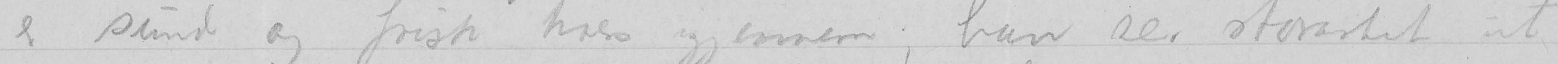er sund og frisk tvers igjennem; han ser storartet ut
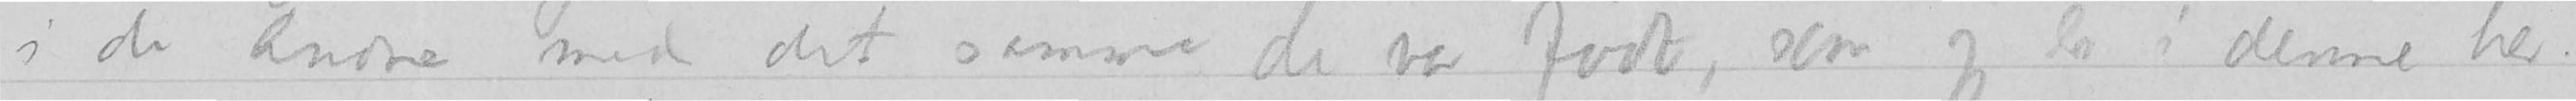i de andre med det samme de var født, som jeg er i denne her.
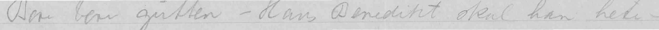Bare bare gutten – Hans Benedikt skal han hete –
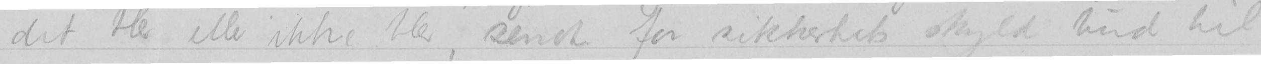det blev eller ikke blev, sendte for sikkerhets skyld bud til
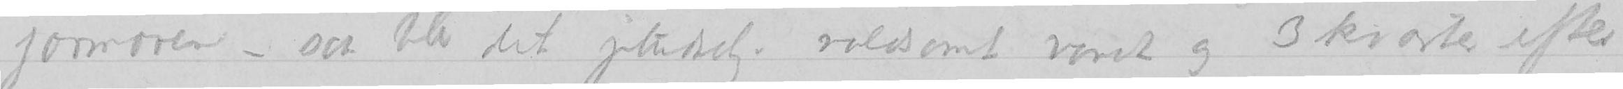jormoren – saa ble det plutselig voldsomt vondt og 3 kvarter efter
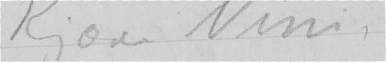Kjære Nini,
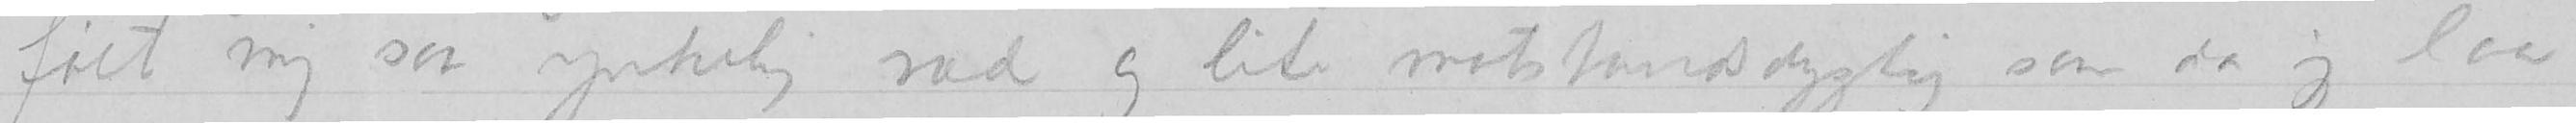følt mig saa ynkelig ræd og lite motstandsdygtig som da jeg laa
